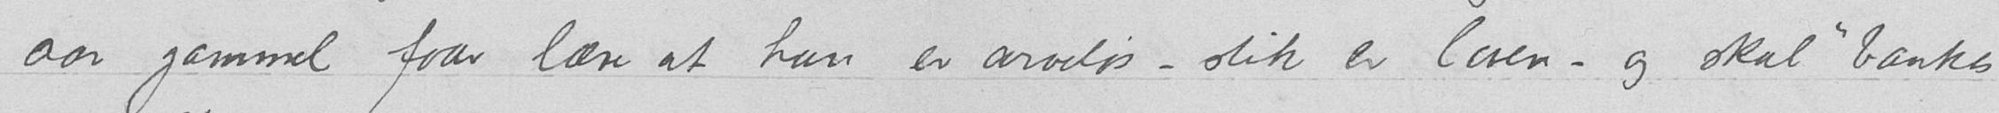aar gammel faar lære at han er arveløs – slik er loven – og skal "bankes
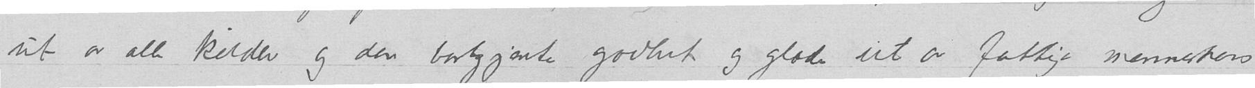ut av alle kilder og den bortgjente godhet og glæde ut av fattige menneskers
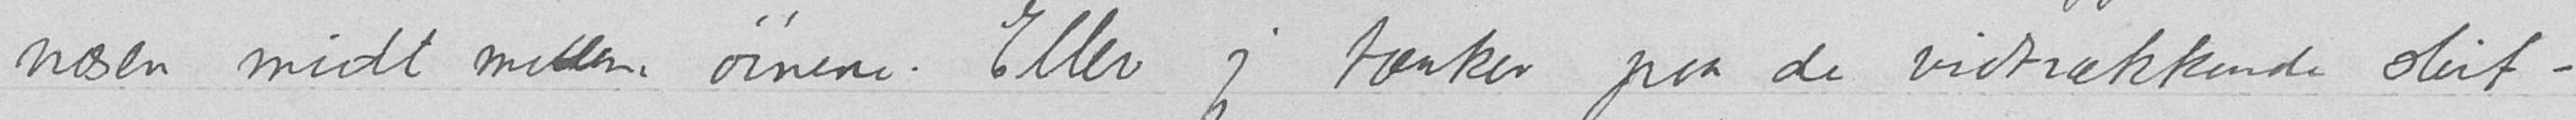næsen midt mellem øinene. Eller jeg tænker paa de vidtrækkende slut-
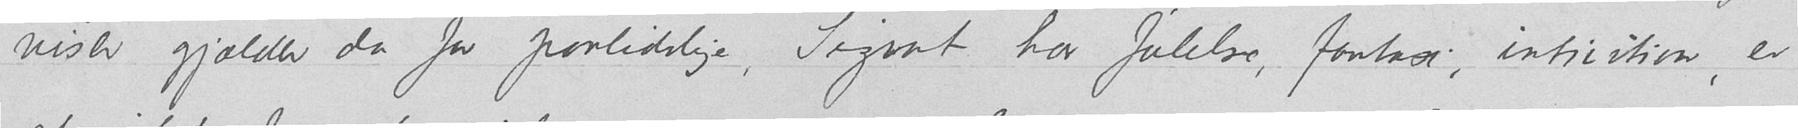viser gjælder da for paalidelige, Sigvat har følelse, fantasi, intuition, er
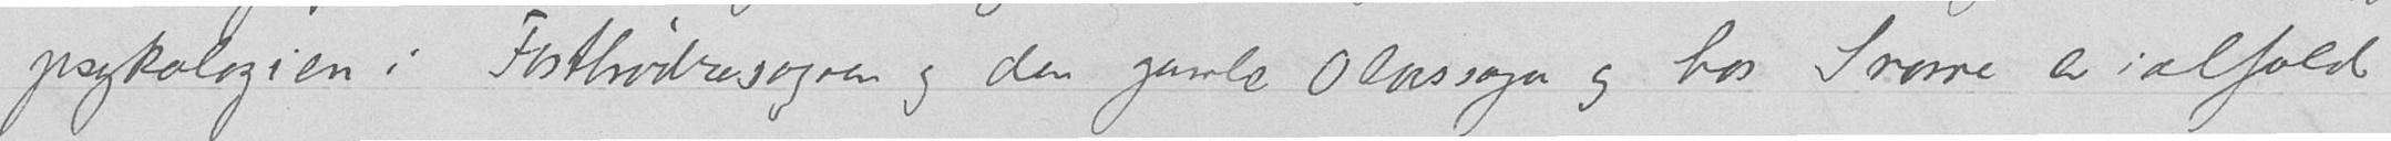psykologien i Fostbrødresagaen og den gamle Olavssaga og hos Snorre er ialfald
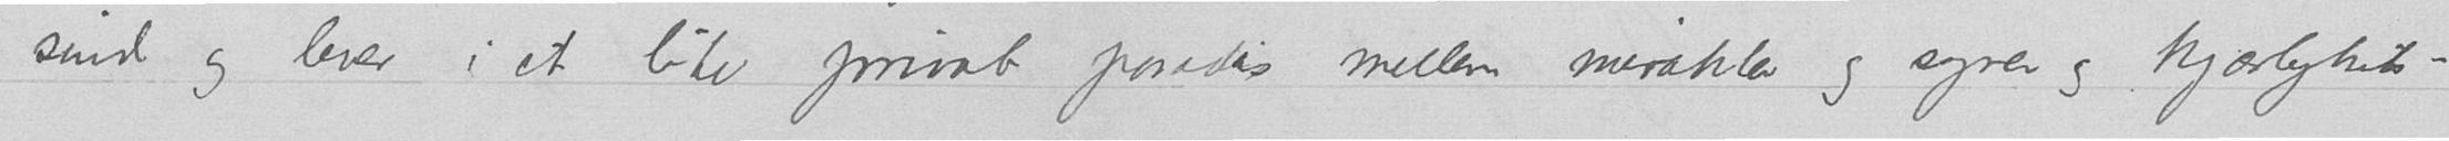sind og lever i et lite privat paradis mellem mirakler og syner og kjærlighets-
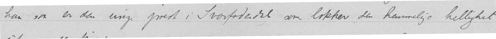han saa er den unge prest i Sværfaderdal som lokker den hemmelige hellighet
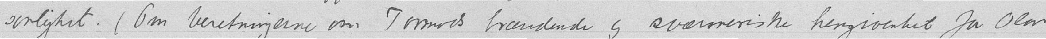sonlighet. (Om beretningerne om Tormods brændende og sværmeriske hengivenhet for Olav
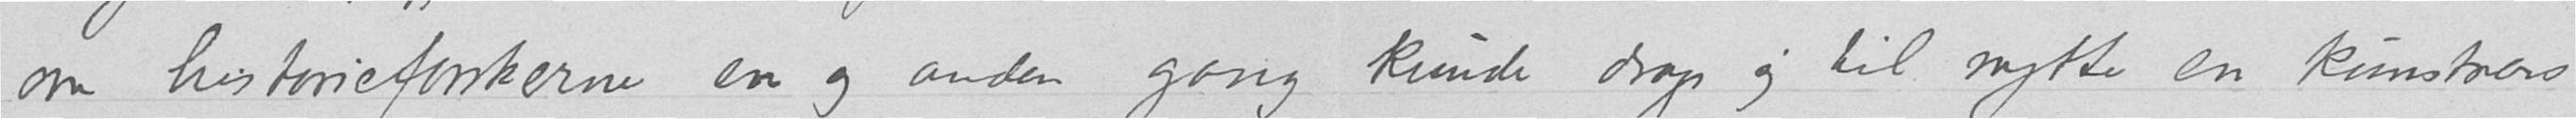om historieforskerne en og anden gang kunde drags sig til nytte en kunstners
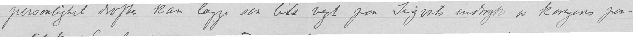personlighet drøftes kan lægge saa lite vegt paa Sigvats indtryk av kongens per-
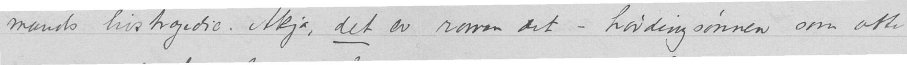mands livstragedie. Akja, det er roman det – høvdingsønnen som otte
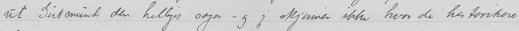ut Gudmund den helliges saga – og jeg skjønner ikke hvor de historikere
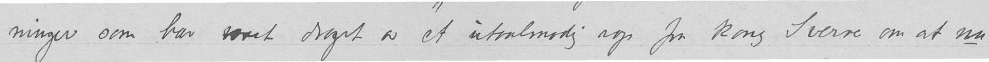ninger som har været draget av et utaalmodig rop fra kong Sverre om at nu
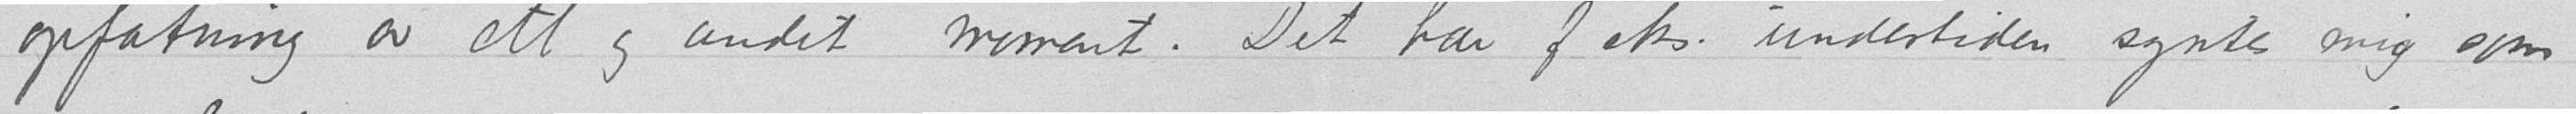opfatning av ett og andet moment. Det har f eks. undertiden syntes mig som
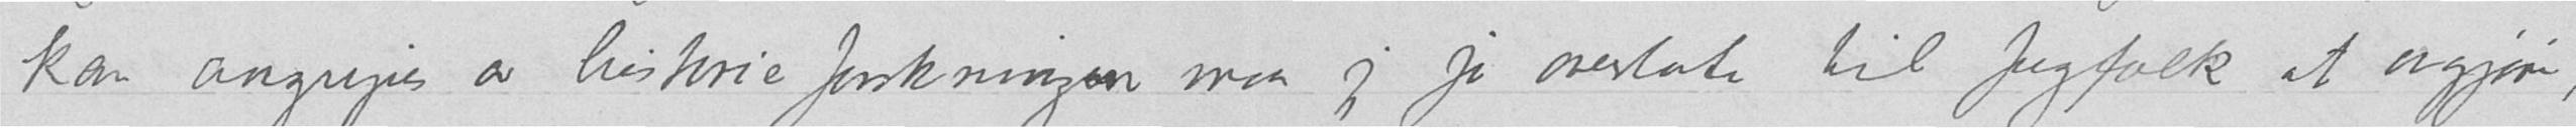kan angripes av historieforskningen maa jeg jo overlate til fagfolk at avgjøre,
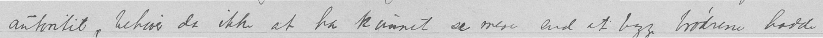autoritet, behøver da ikke at ha kunnet se mere end at begge brødrene hadde
